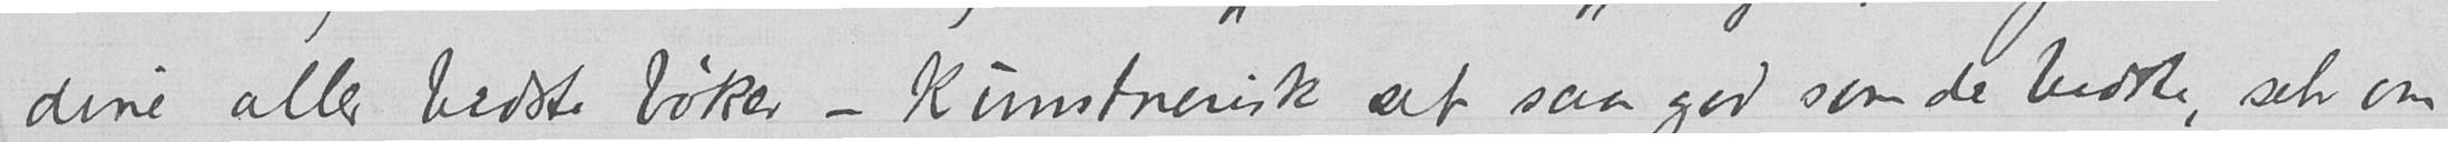dine aller bedste bøker - kunstnerisk set saa god som de bedste, selv om
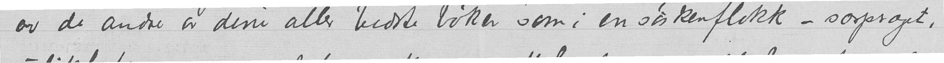av de andre av dine aller bedste bøker som i en søskenflokk - særpræget,
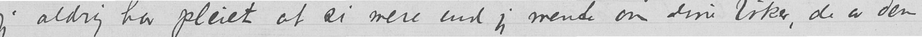jeg aldrig har pleiet at si mere end jeg mente om dine bøker, de av dem
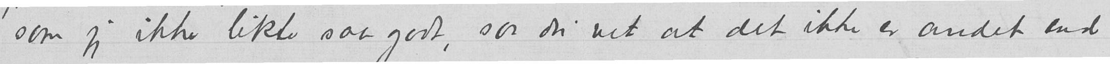som jeg ikke likte saa godt, saa du vet at det ikke er andet end
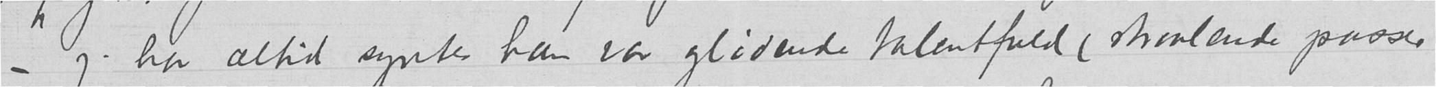– jeg har altid syntes han var glødende talentfuld (straalende passer
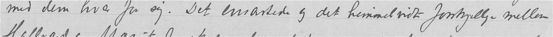med dem hver for sig. Det ensartede og det himmelvidt forskjellige mellem
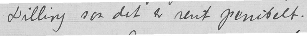Dilling saa det er rent penibelt.
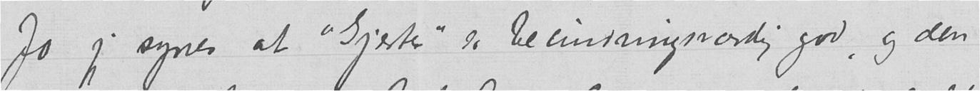Jo jeg synes at "Gjester" er beundringsværdig god, og den
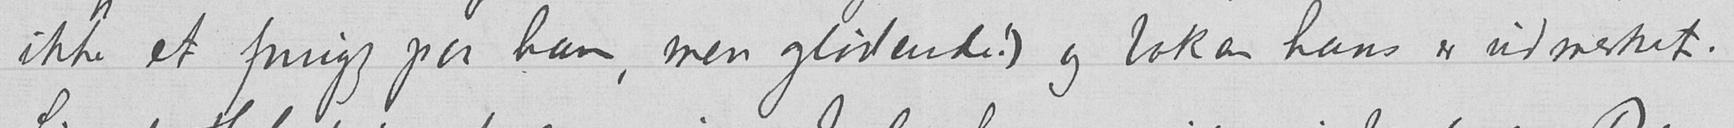ikke et fnugg paa ham, men glødende!) og boken hans er udmerket.
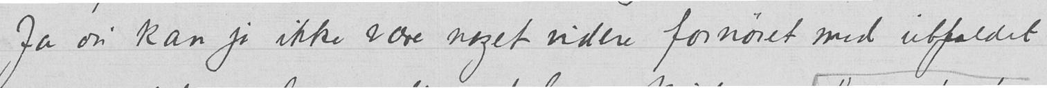Ja du kan jo ikke være noget videre fornøiet med utfaldet
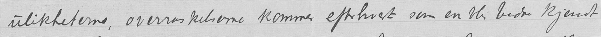ulikheterne, overraskelserne kommer efterhvert som en blir bedre kjendt
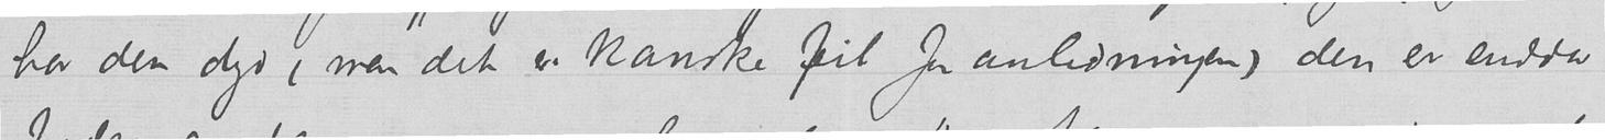har den dyd (men det er kanske feil for anledningen) den er endda
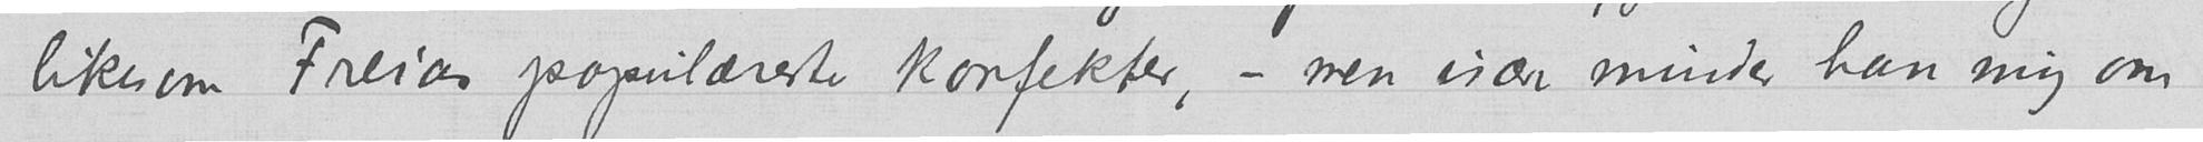likesom Freias populæreste konfekter, – men især minder han mig om
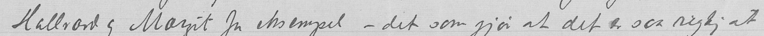Hallvard og Margit for eksempel – det som gjør at det er saa rigtig at
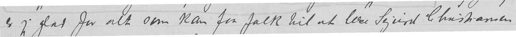er jeg glad for alt som kan faa folk til at læse Sigurd Christiansen
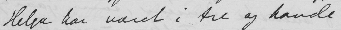Helga har været i tre og havde
